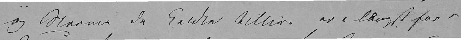og Storme de kaldte tillive ere længst
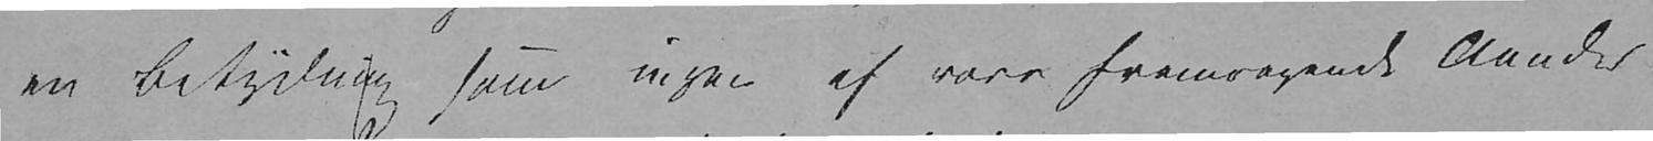en Betydning som ingen af vore fremragende Aander
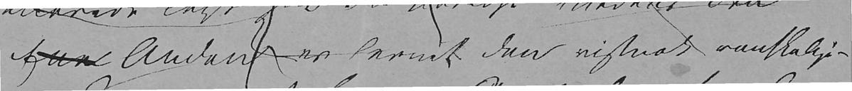Ene Anden er levnet den vistnok vanskeligere
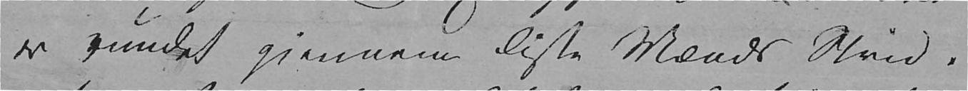er vundet gjennem disse Mænds Strid,
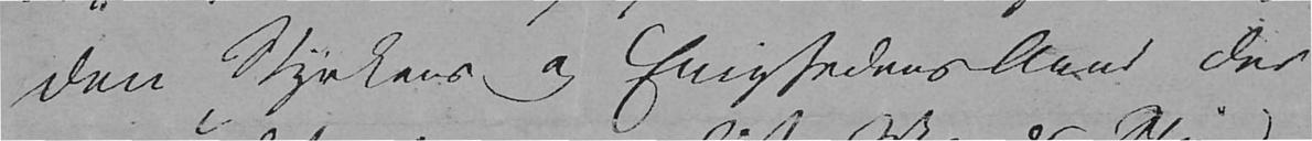den Styrkens og Enighedens Aand der
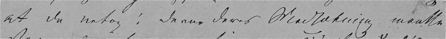at de netop i denne deres Modsætning maatte
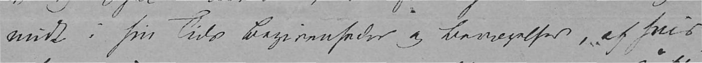midt i sin Tids Begivenheder og Bevægelser, af hvis
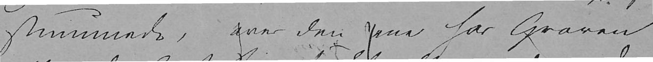forstummede, over den ene har Graven
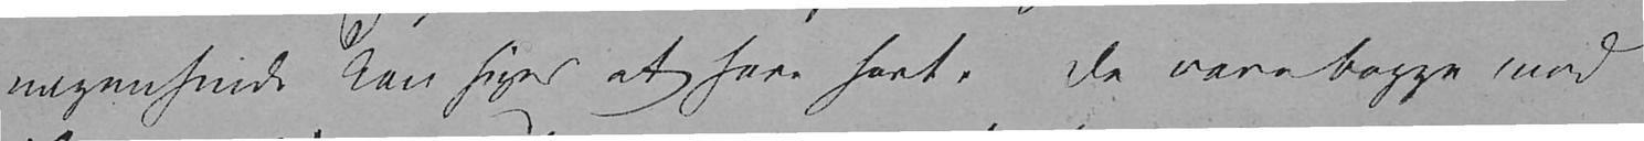nogensinde kan siges at have havt. De vare begge med
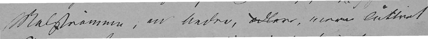Malstrømme, en bedre, ædlere, mere luttret
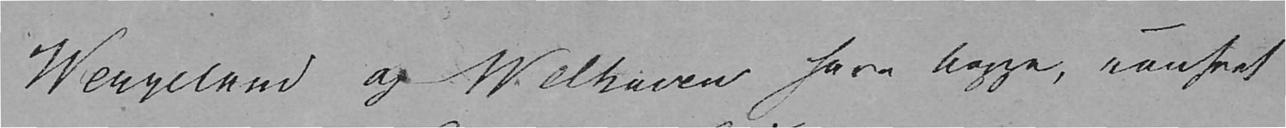Wergeland og Welhaven have begge, uanseet
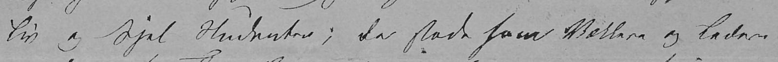Liv og Sjel Studenter; de stode som Vækkere og Ledere
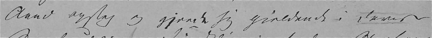Aand opsteg og gjorde sig gjeldende i deres
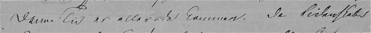Denne Tid er allerede kommen. De Lidenskaber
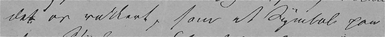det os vakkert, som et Symbol paa
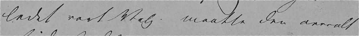ledet vort Valg. maatte den overalt
